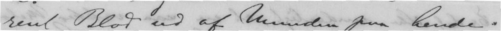rent Blod ud af Munden paa hende.
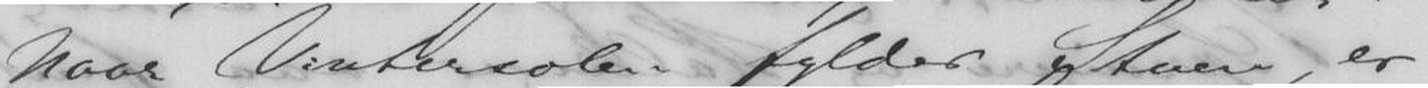Naar vintersolen fylder Stuen, er
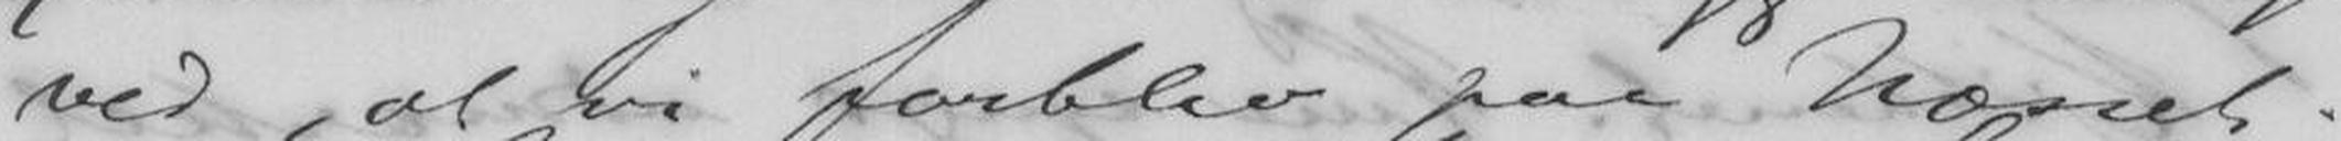ved, at vi forblev paa Næsset.
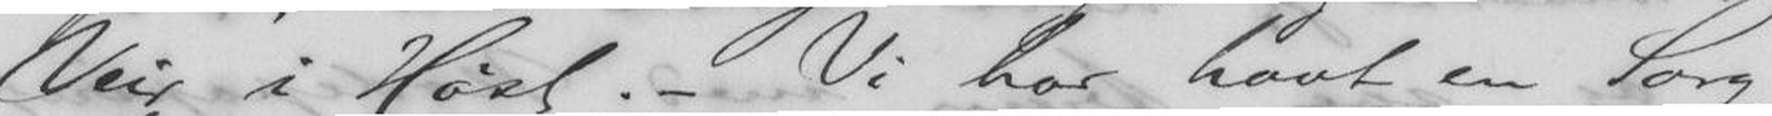Veir i Høst. - Vi har havt en Sorg
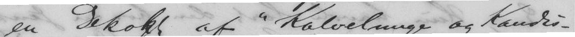en Dekokt af "Kalvelunge og Kandis-
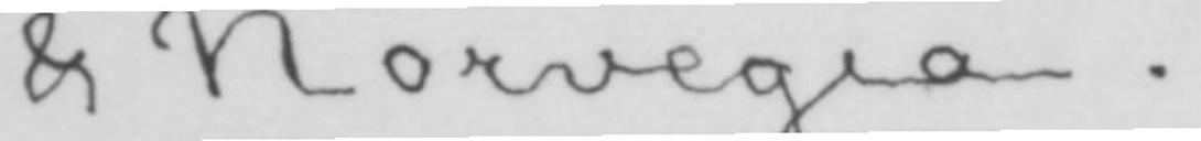& Norvegia.
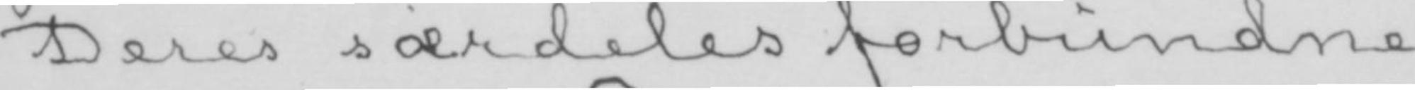Deres særdeles forbundne
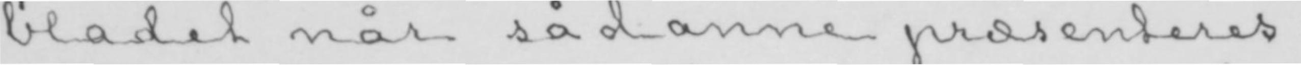bladet når sådanne præsenteres.
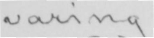varing.
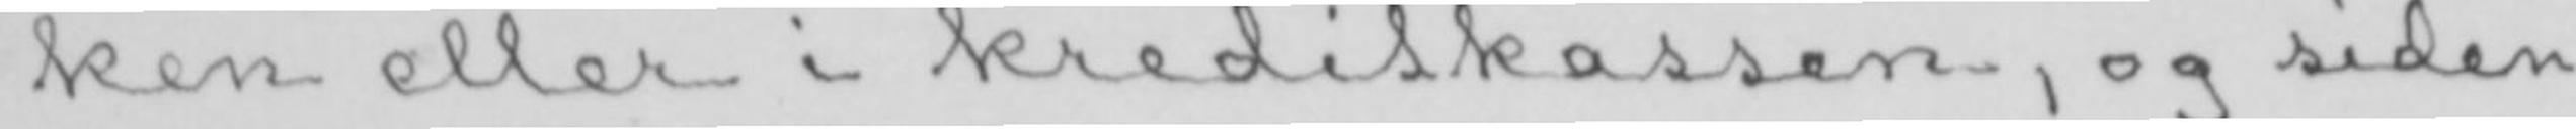ken eller i kreditkassen, og siden
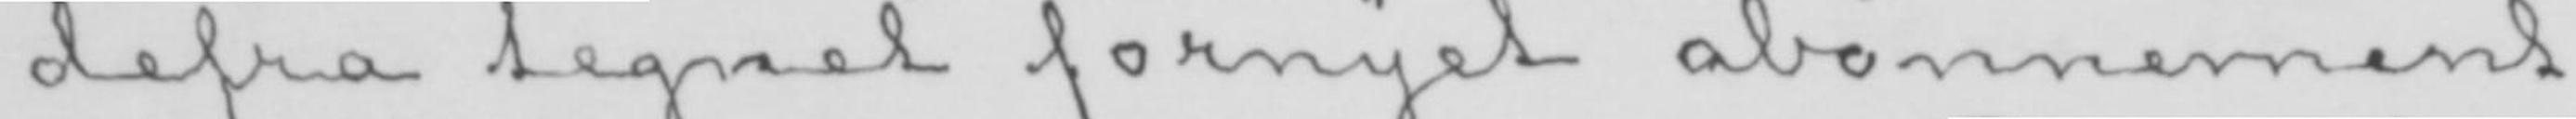defra tegnet fornyet abonnement
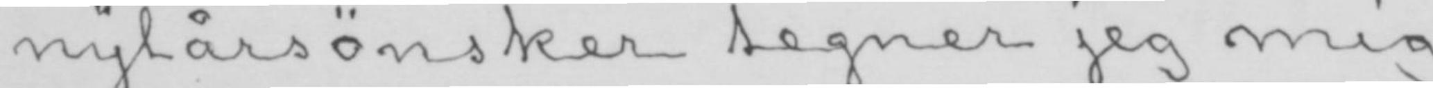nytårsønsker tegner jeg mig
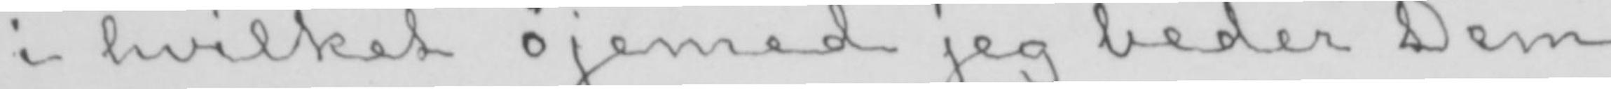i hvilket øjemed jeg beder Dem
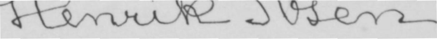Henrik Ibsen.
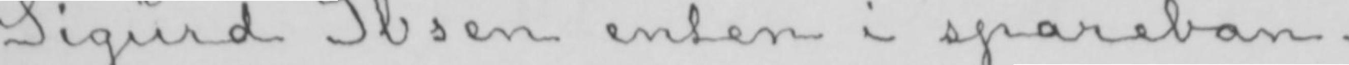Sigurd Ibsen enten i spareban-
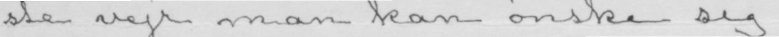ste vejr man kan ønske sig;
